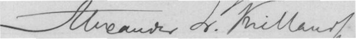Alexander L. Kielland
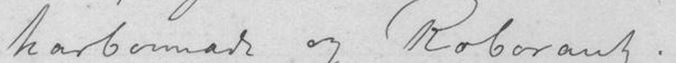karbonade og Roborantg.
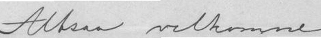Altsaa velkomne
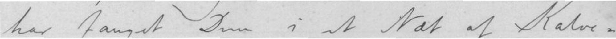har fanget Dem i et Næt af Kalve-
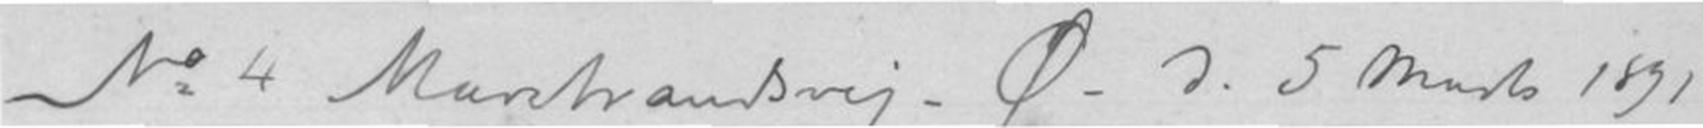No 4 Marstrandsvej Ø- d. 5 Marts 1891
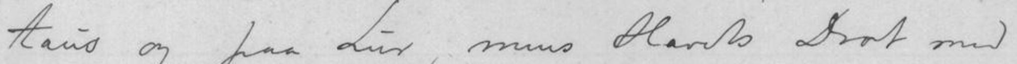taus og paa Lur, mens med
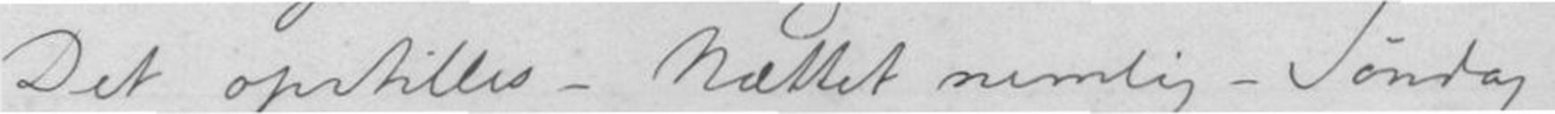Det opstilles - Nættet nemlig - Søndag
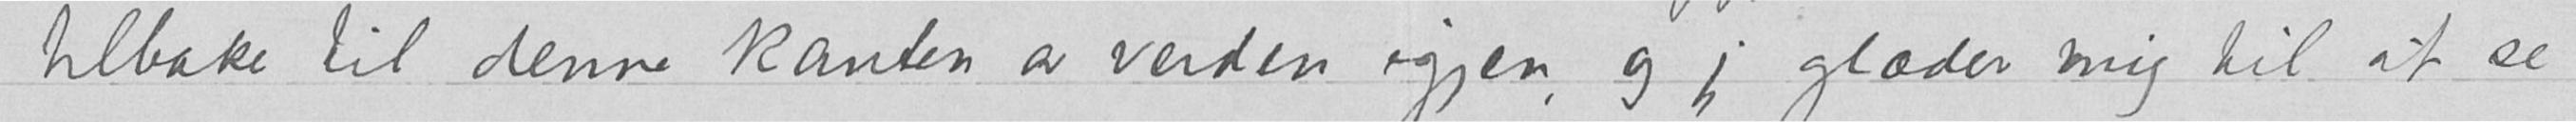tilbake til denne kanten av verden igjen, og jeg glæder mig til at se
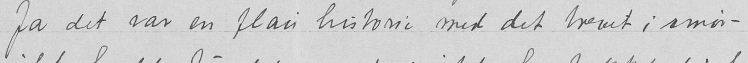Ja det var en flau historie med det brevet i smør-
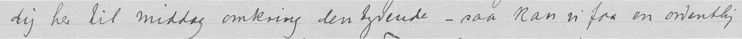dig her til middag omkring den tyvende – saa kan vi faa en ordentlig
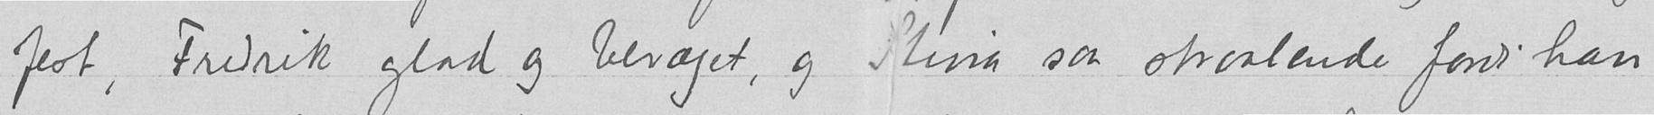fest, Fredrik glad og bevæget, og Stina saa straalende fordi han
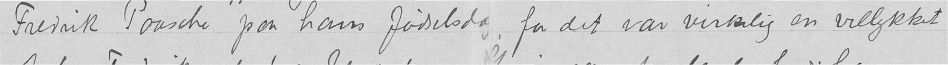Fredrik Paasche paa hans fødselsdag, for det var virkelig en vellykket
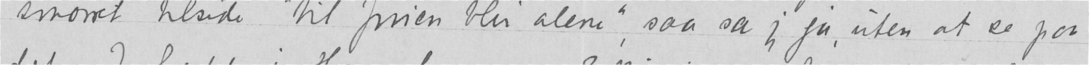smørret tilside «til fruen blir alene», saa sa jeg ja, uten at se paa
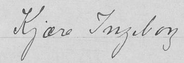Kjære Ingeborg
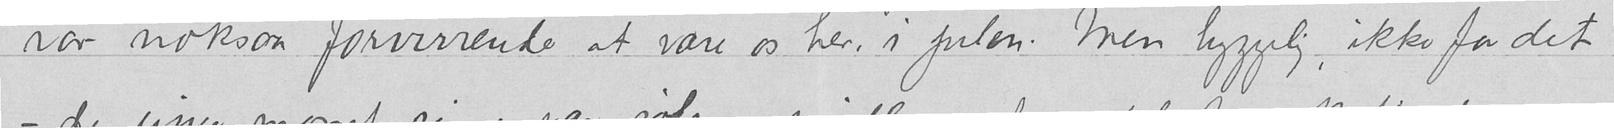var noksaa forvirrende at være os her, i julen. Men hyggelig, ikke for det
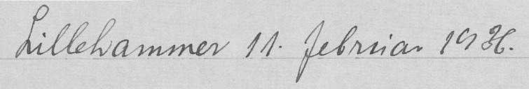Lillehammer 11. februar 1936.
